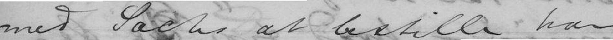med Sachs at bestille har
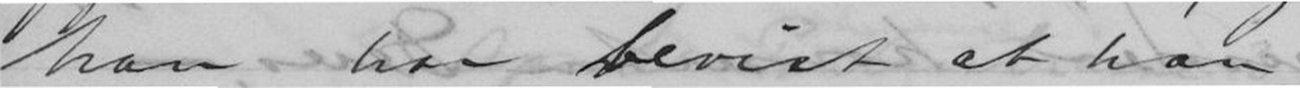han har bevist at han
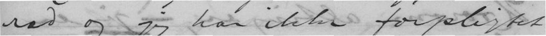ræd og jeg har ikke forpligtet
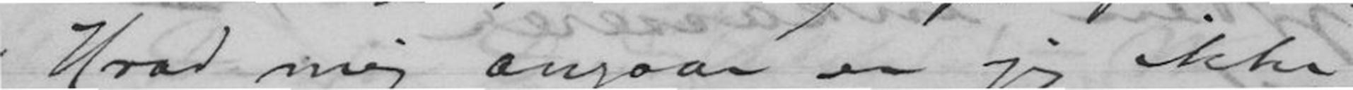- Hvad mig angaar er jeg ikke
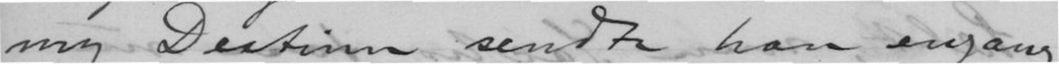my Destinn sendte han engang
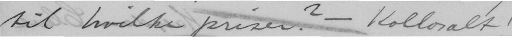og til hvilke priser? - Kollosalt!
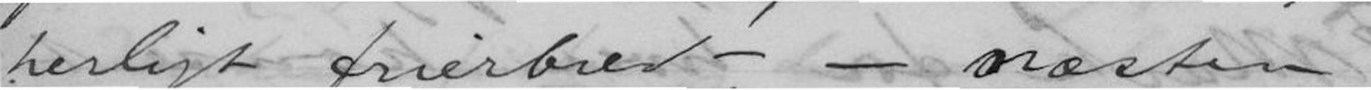herligt frierbrev, - - næsten
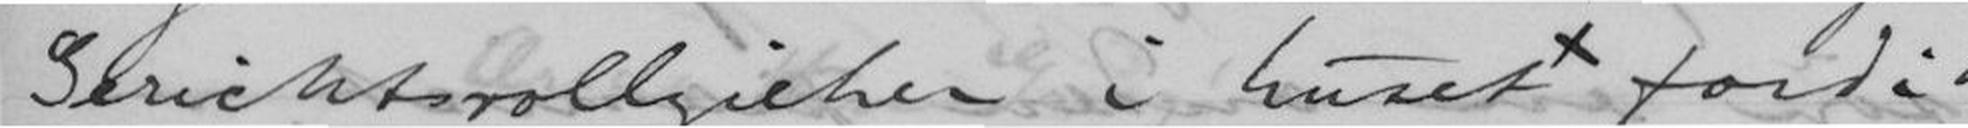Gerichtsrollziehen i Husetx fordi
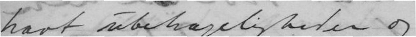havt ubehageligheder og
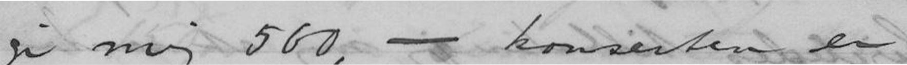gi mig 500, - konserten er
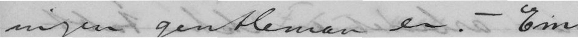ingen gentleman er. - Em-
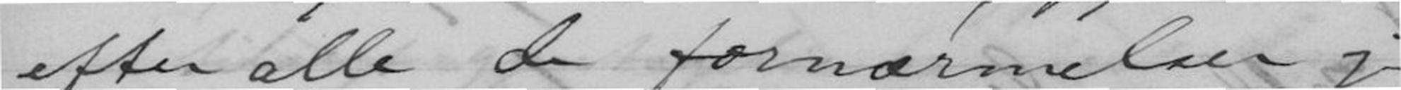efter alle de fornærmelser jeg
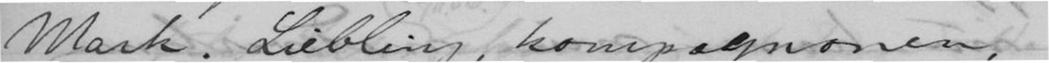Mark. Liebling, kompagnonen,
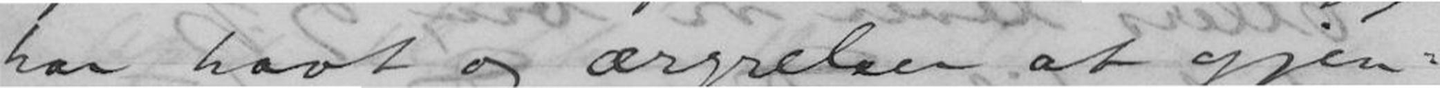har havt og ærgrelser at gjen-
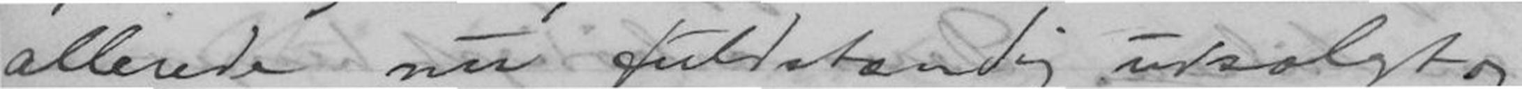allerede nu fuldstændig udsolgt
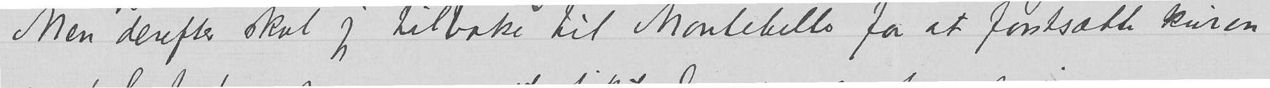Men derefter skal jeg tilbake til Montebello for at fortsætte kuren
